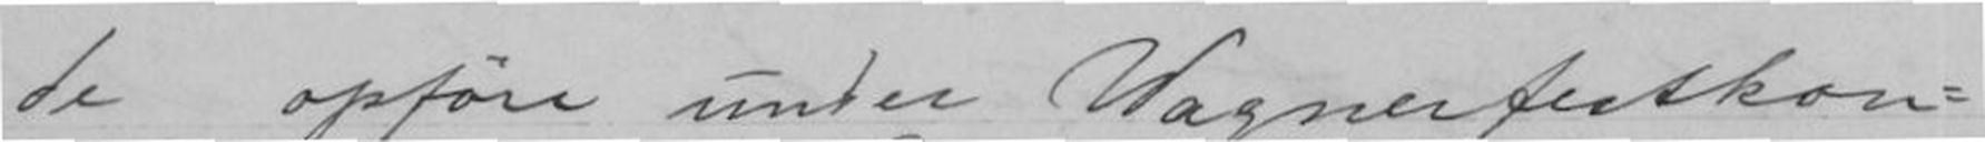de opføre under Wagnerfestkon-
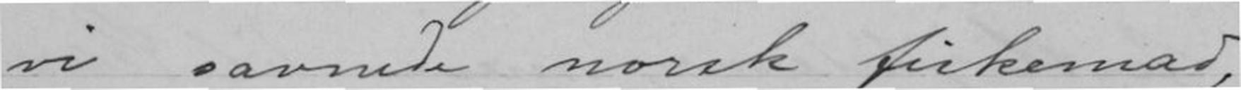vi savnede norsk fiskemad,
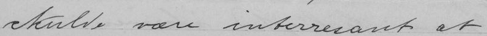skulde være interresant at
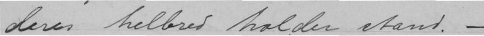deres helbred holder stand. -
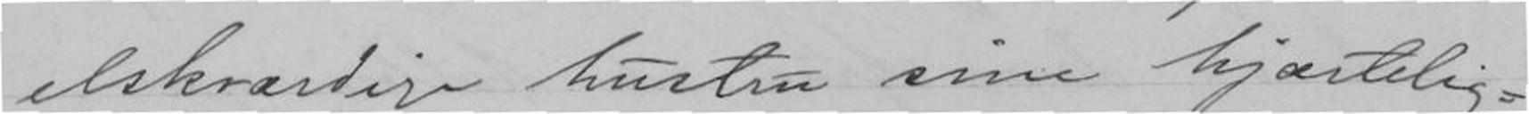elskværdige hustru sine hjærtelig-
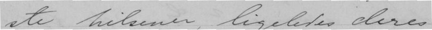ste hilsener, ligeledes deres
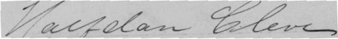Halvdan Cleve
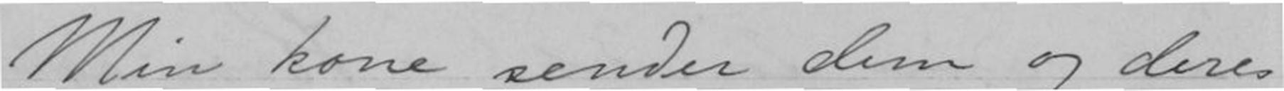Min kone sender dem og deres
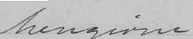hengevne
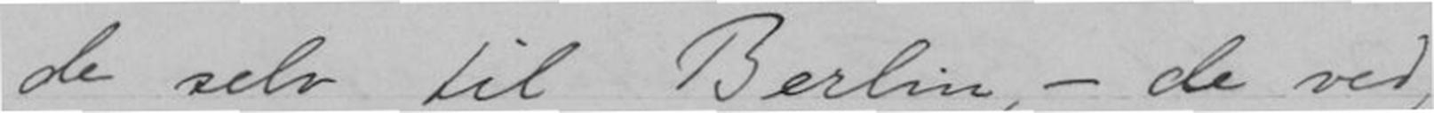de selv til Berlin, - de ved
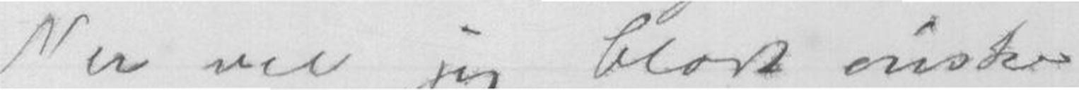Nu vil jeg blodt ønske
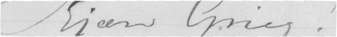Kjære Grieg!
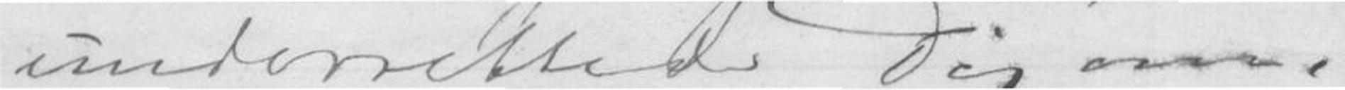underrettede Dig om, sendtes
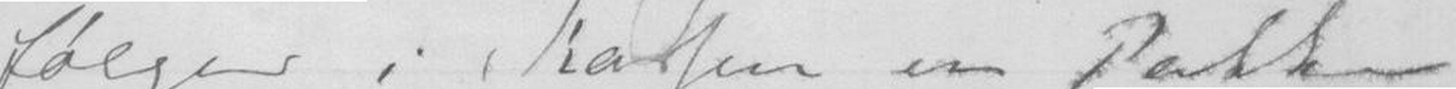følger i Kassen en Pakke
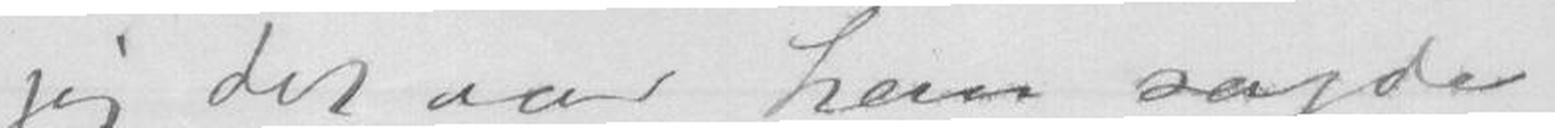jeg det var han sagde -
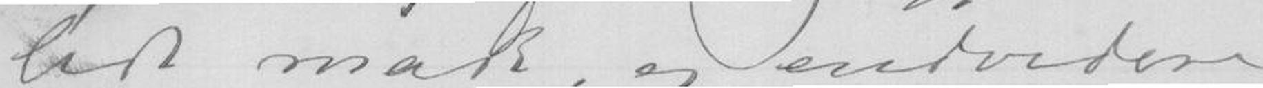lidt madt, og endvidere
